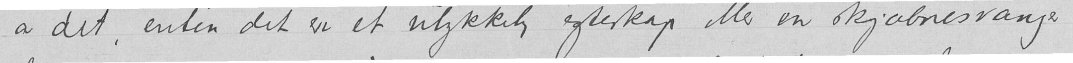av det, enten det er et ulykkelig egteskap eller en skjæbnesvanger
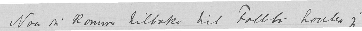Naar du kommer tilbake til Follebu haaber jeg
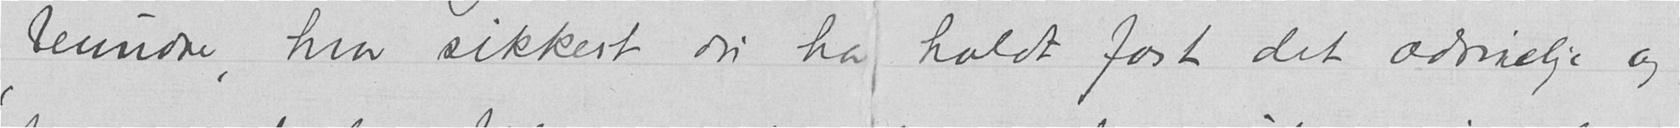jeg beundre, hvor sikkert du har holdt fast det ædruelige og
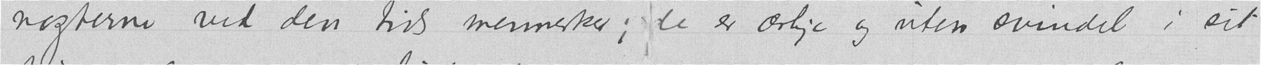nøgterne ved den tids mennesker; de er ærlige og uten svindel i sit
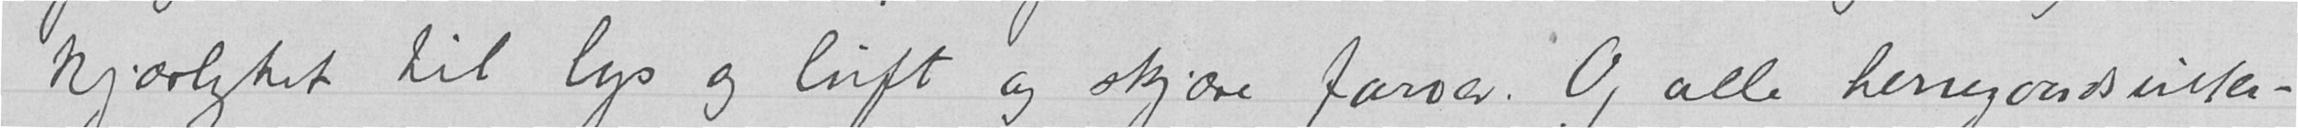kjærlighet til lys og luft og skjære farver. Og alle herregaardsinter-
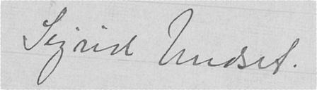Sigrid Undset.
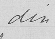din
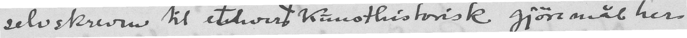selvskreven til ethvert kunsthistorisk gjøremål her
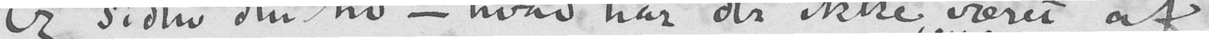Og siden den tid — hvad har der ikke været af
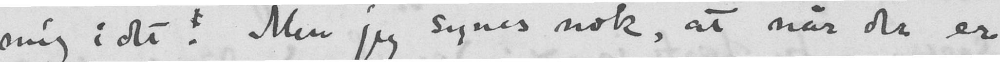mig i det! Men jeg synes nok, at når der er
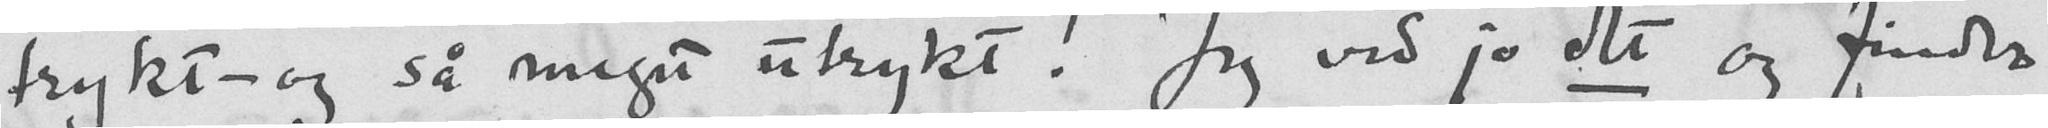trykt - og så meget utrykt! Jeg ved jo det og finder
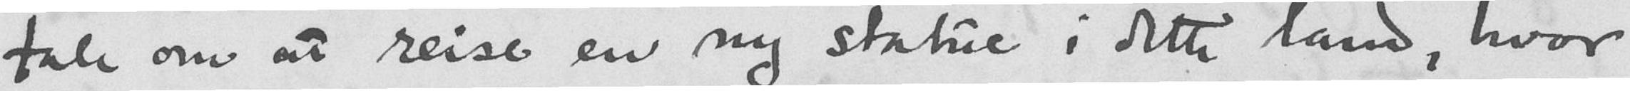tale om at reise en ny statue i dette land, hvor
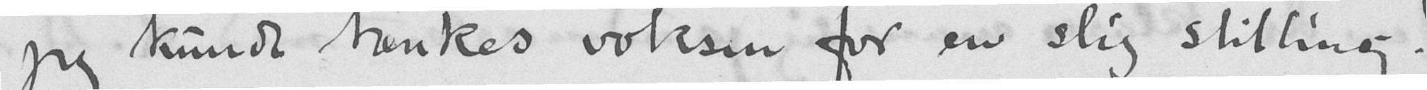jeg kunde tænkes voksen for en slig stilling !
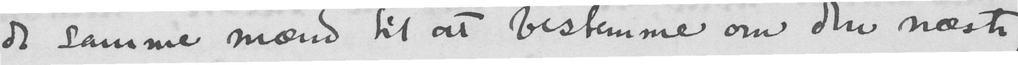de samme mænd til at bestemme om den næste,
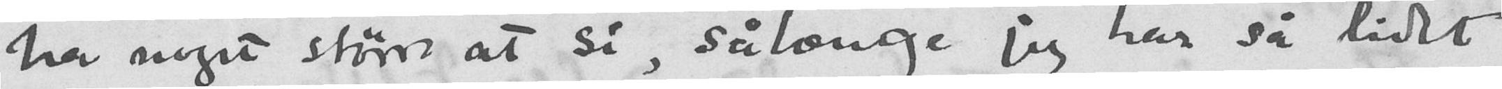ha noget større at si, sålænge jeg har så lidet
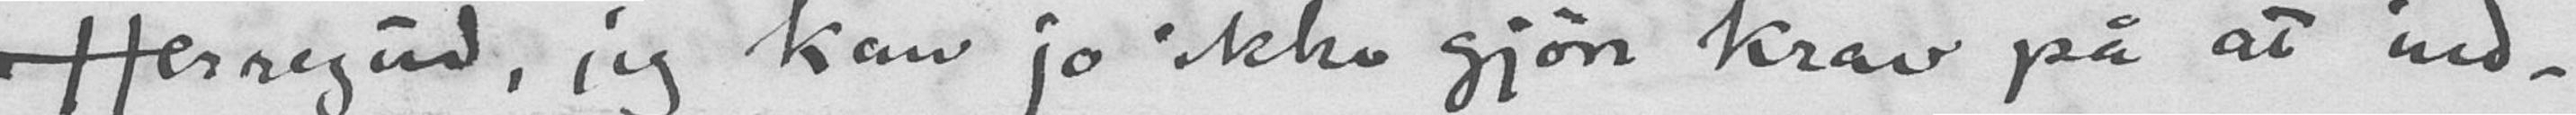Herregud, jeg kan jo ikke gjøre krav på at ind-
